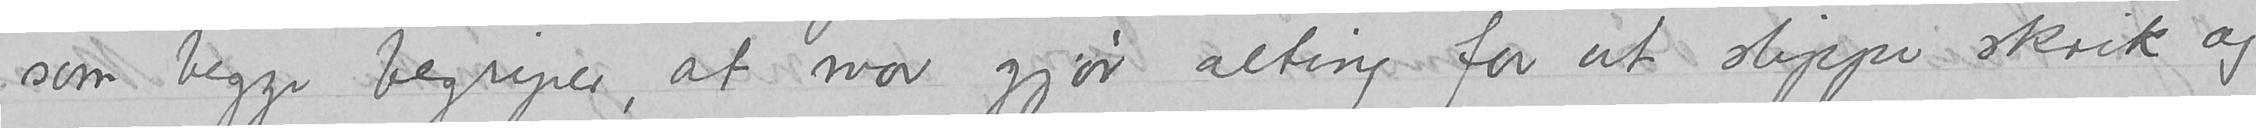som begge begriper, at mor gjør alting for at slippe skrik og
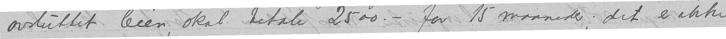avsluttet leien, skal betale 2500.- for 15 maaneder; det er ikke
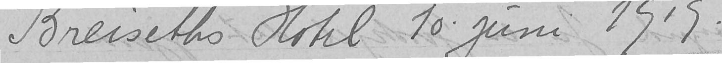Breiseths Hotel 10. juni 1919.
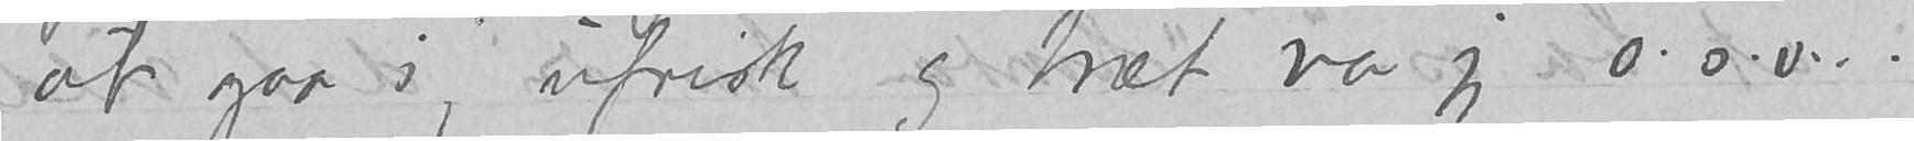at gaa i, ufrisk og træt var jeg o.s.v.
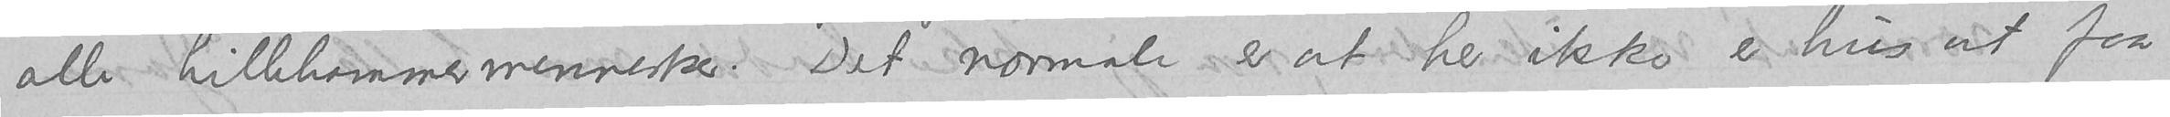alle Lillehammermennesker. Det normale er at her ikke er hus at faa
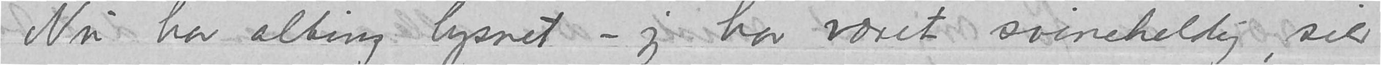Nu har alting lysnet – jeg har været svineheldig, sier
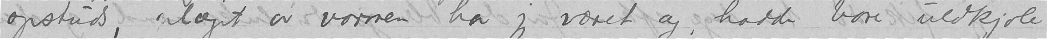opstuds, plaget av varmen har jeg været og, hadde bare uldkjole
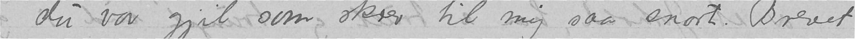du var gjil som skrev til mig saa snart. Brevet
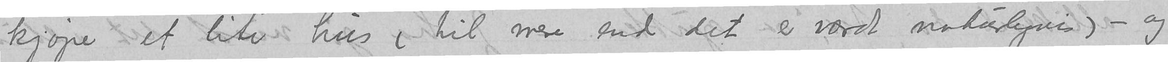kjøpe et lite hus (til mere end det er værdt naturligvis) – og
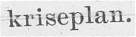kriseplan.
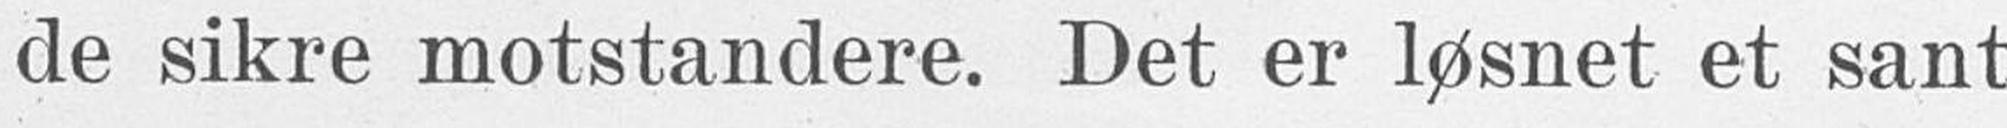de sikre motstandere. Det er løsnet et sant
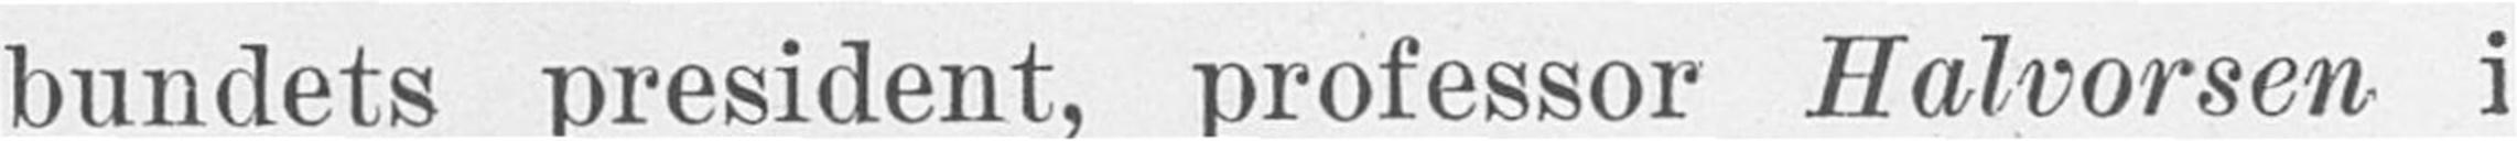bundets president, professor Halvorsen i
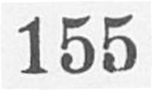155
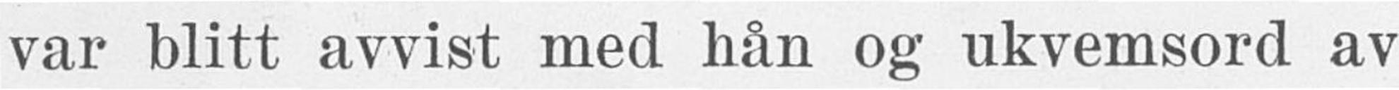var blitt avvist med hån og ukvemsord av
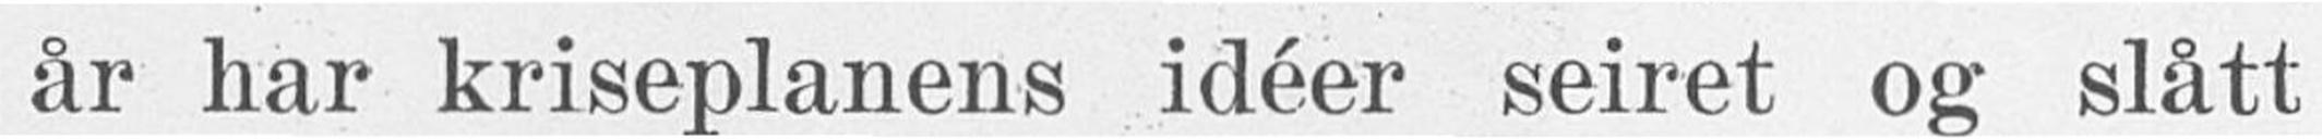år har kriseplanens idéer seiret og slått
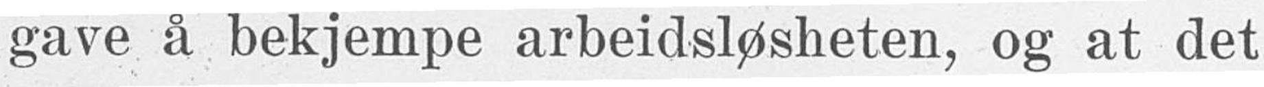gave å bekjempe arbeidsløsheten, og at det
